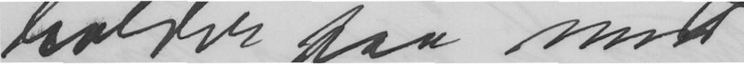holder paa med
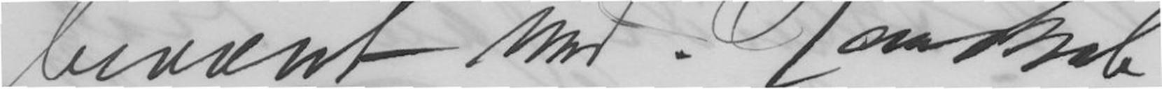bevænt med. Romskab
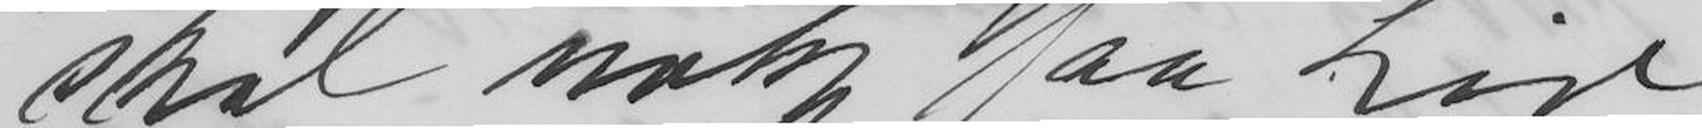skal netop faa Liv
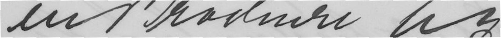en Brochure jeg
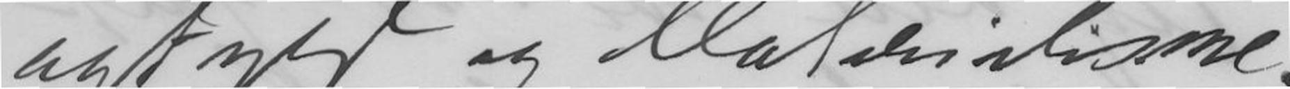og Fyld og Malerielisme.
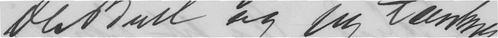Ole Bull og jeg tænker
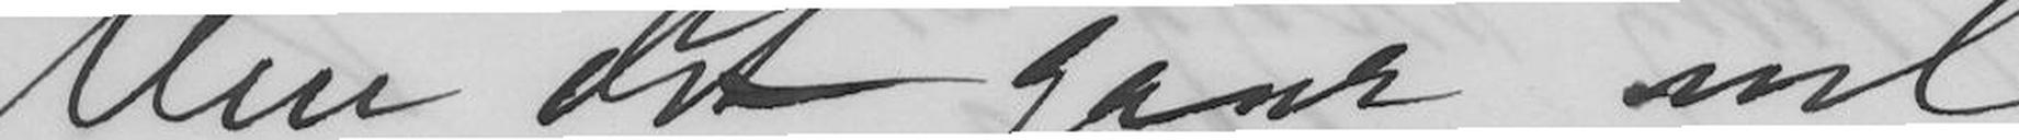Men det gaar vel
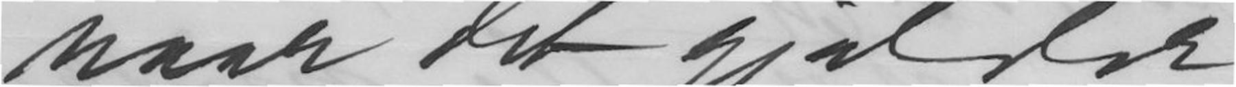naar de gjælder
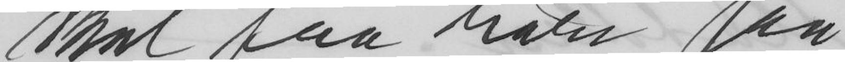skal faa høre saa
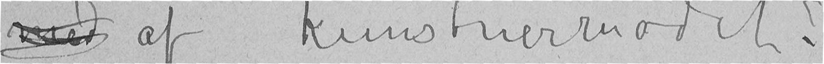med af kunstnermødet?
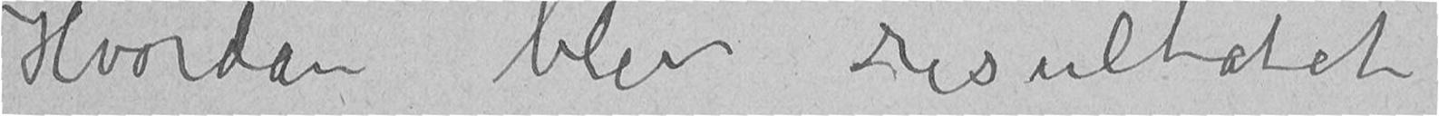Hvordan blev resultatet
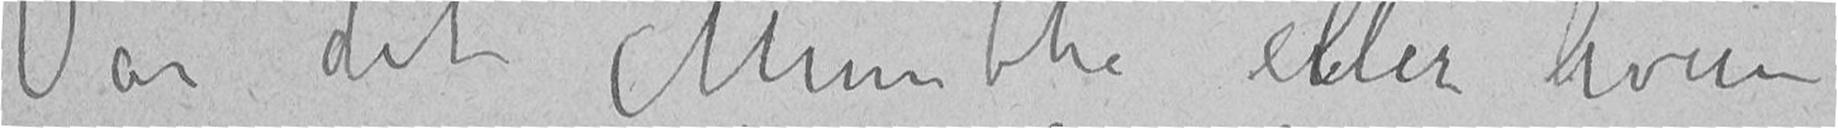Var det Munthe eller hvem
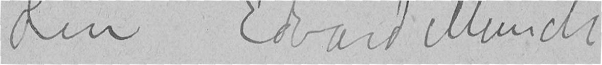din Edvard Munch
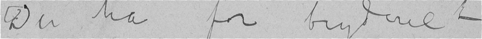Du ha for bryderiet
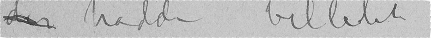der hadde billedet?
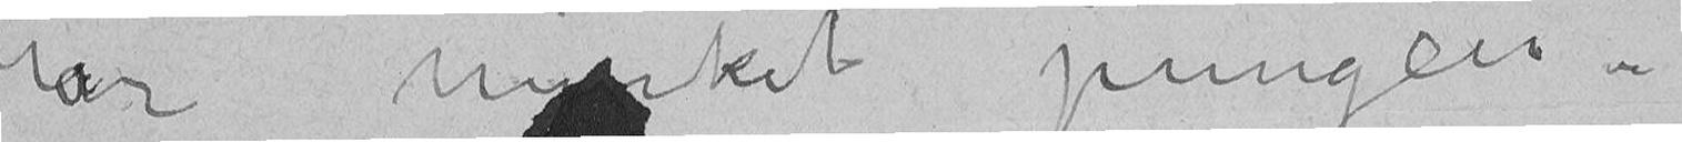har minket pengene –
